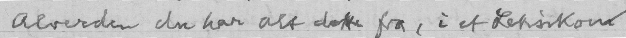Alverden du har alt dette fra, i et Leksikon
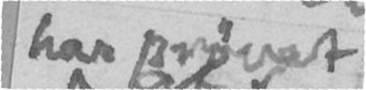har prøvet
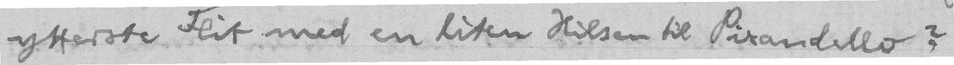ytterste Flit med en liten Hilsen til Pirandello?
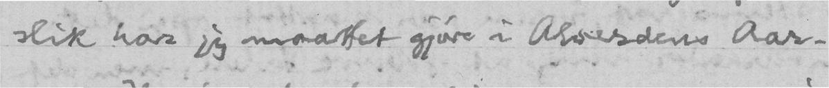slik har jeg maattet gjøre i Alverdens Aar.
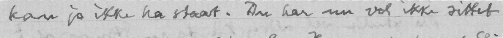kan jo ikke ha staat. Du har nu vel ikke sittet
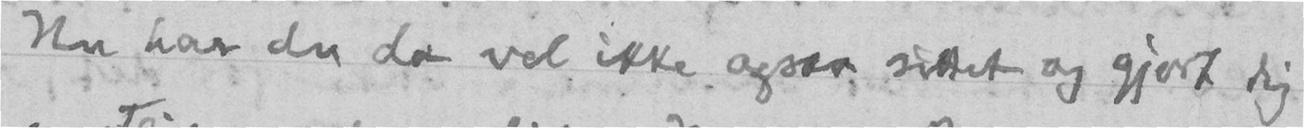Nu har du da vel ikke ogsaa sittet og gjort dig
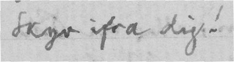Skyv ifra dig!
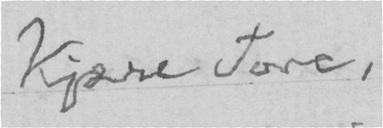Kjære Tore,
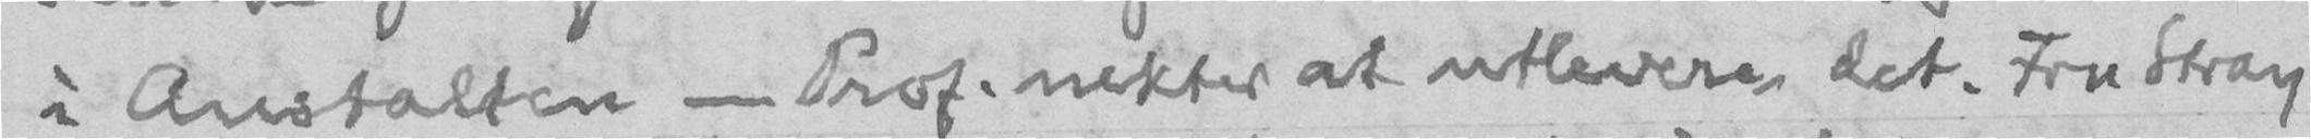i Anstalten - Prof. nekter at utlevere det. Fru Stray
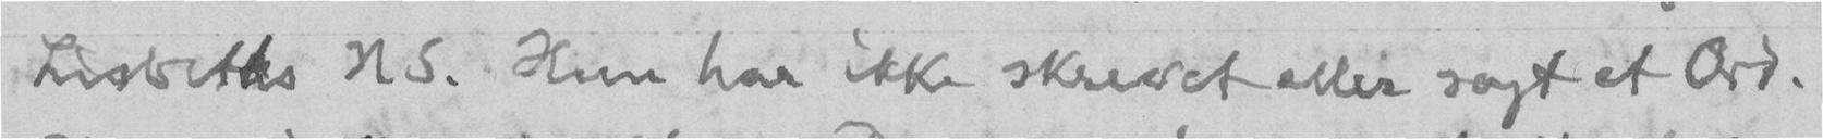Lisbeths NS. Hun har ikke skrevet eller sagt et Ord.
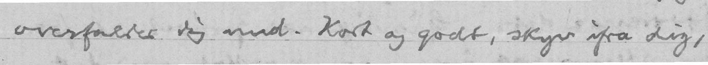overfalder dig med. Kort og godt, skyv ifra dig,
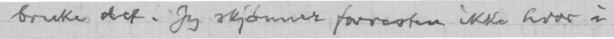bruke det. Jeg skjønner forresten ikke hvor i
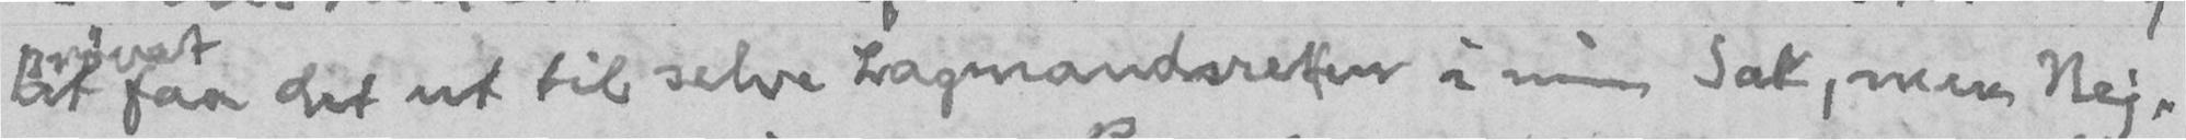at faa det ut til selve Lagmandsretten i min Sak, men Nej.
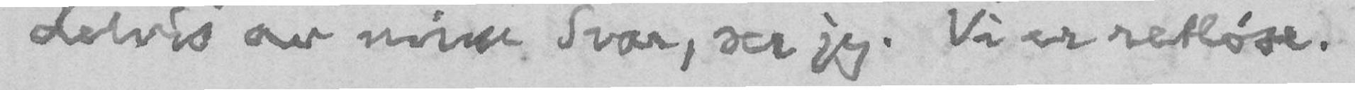delvis av mine Svar, ser jeg. Vi er retløse.
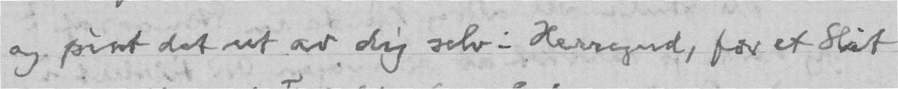og pint det ut av dig selv. Herregud, for et Slit
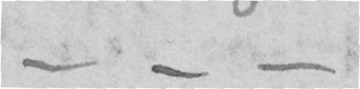- - -
